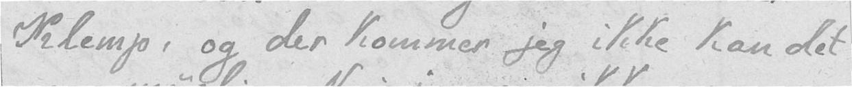Klemp, og der kommer jeg ikke kan det
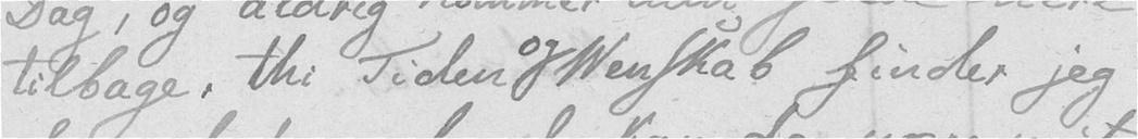tilbage, thi Tiden og Venskab finder jeg
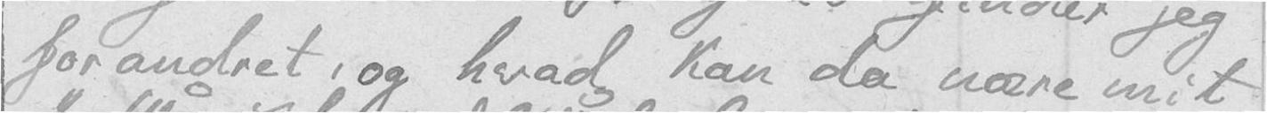forandret, og hvad kan da nære mit
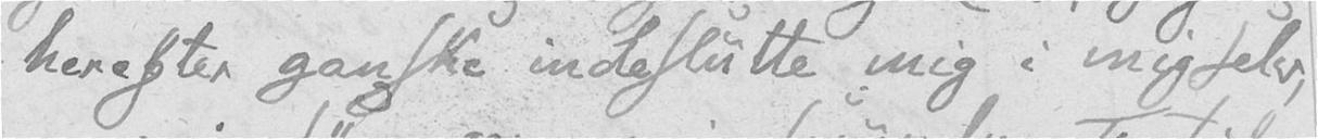herefter ganske indeslutte mig i mig selv,
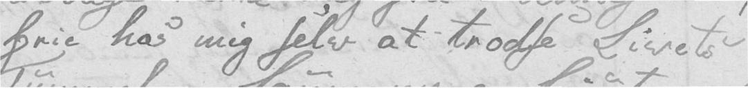frie hos mig selv at trodse Livets
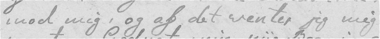mod mig, og af det venter jeg mig
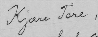Kjære Tore,
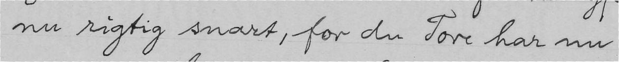nu rigtig snart, for du Tore har nu
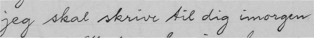jeg skal skrive til dig imorgen
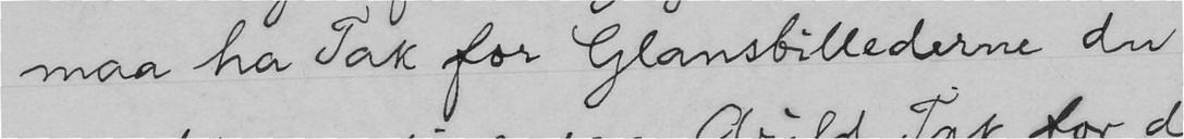maa ha Tak for Glansbillederne du
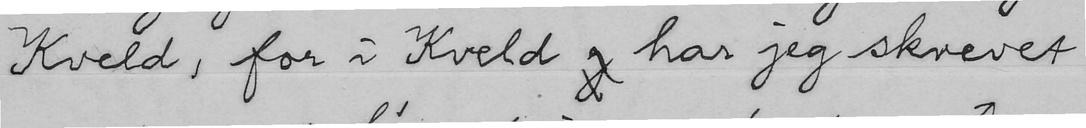Kveld, for i Kveld og har jeg skrevet
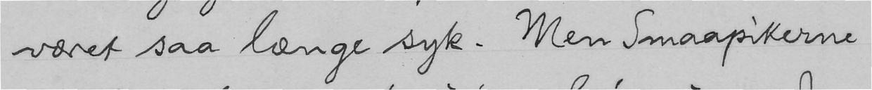været saa længe syk. Men Smaapikerne
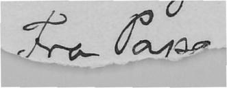Fra Papa
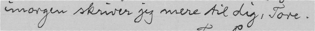imorgen skriver jeg mere til dig, Tore.
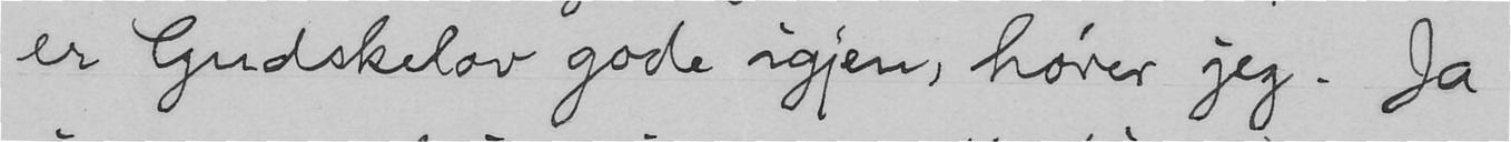er Gudskelov gode igjen, hører jeg. Ja
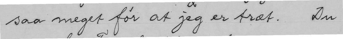saa meget før at jeg er træt. Du
